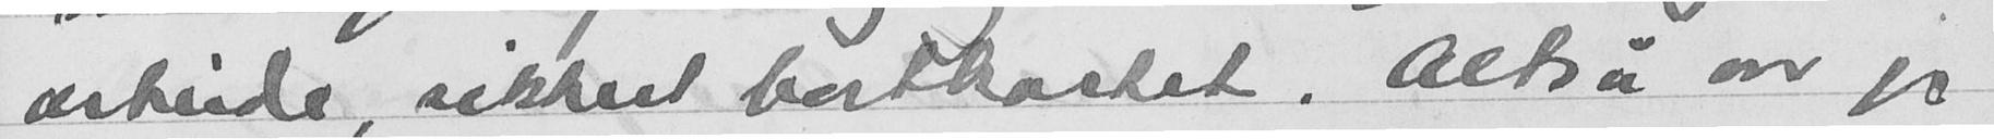arbeide, sikkert bortkastet. Altså var jeg
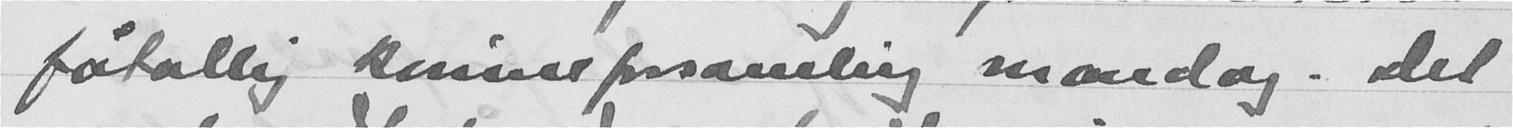fåtallig kvinneforsamling mandag. Det
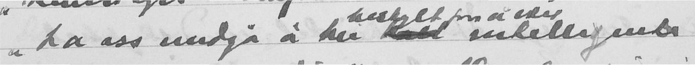" La oss undgå å bli beskylt for å virke intelligente
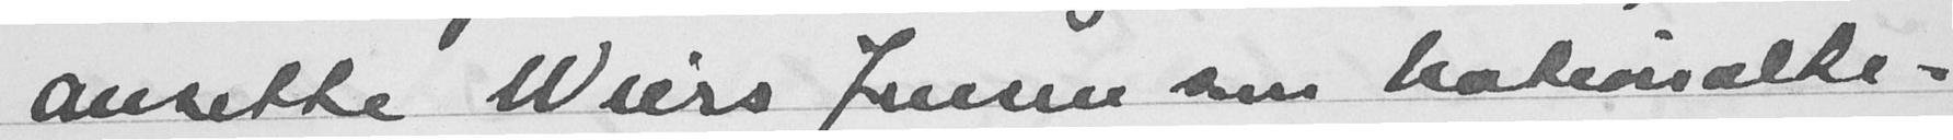ansette Wiers Jensen som Nationalthe-
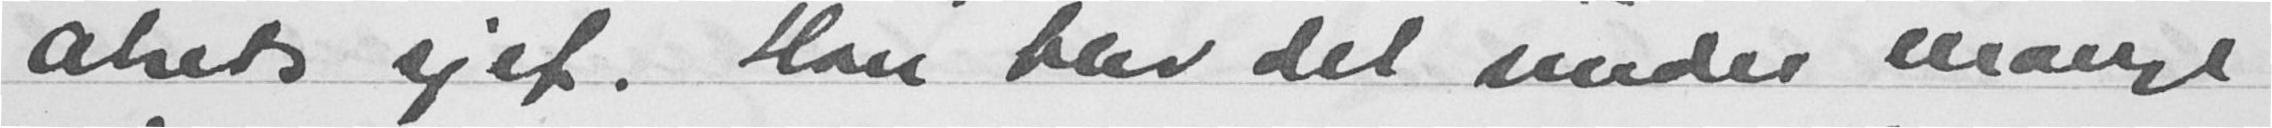aterets sjef. Han blev det under mange
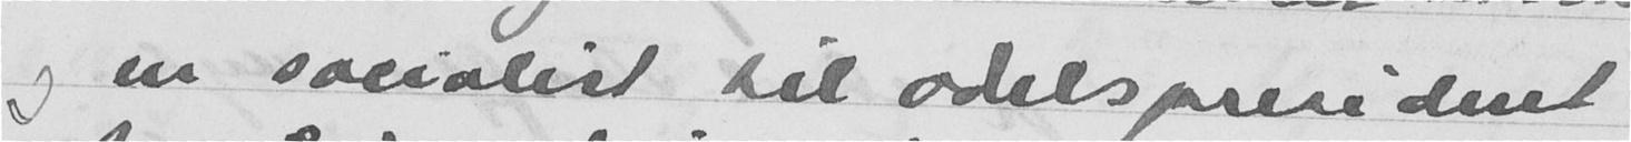og en socialist til adelspresident -
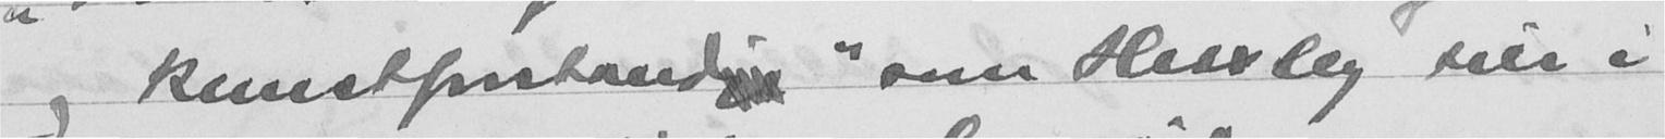og kunstforstand som Heiberg sier i
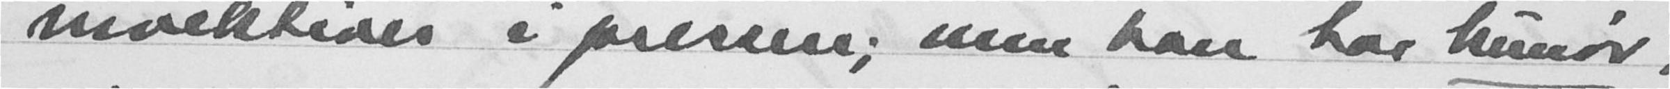invektiver i pressen; men han har humør,
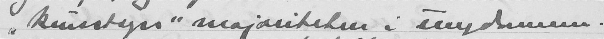"kunstsyn" majoriteten i ungdomen.
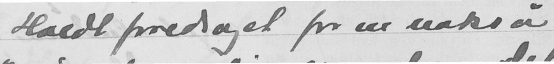Holdt foredraget for en nokså
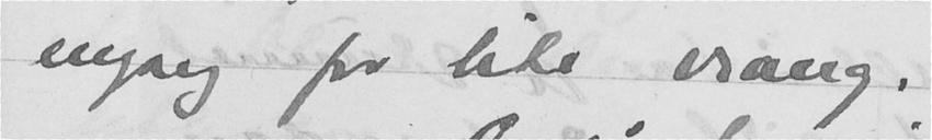engang for lite vrang.
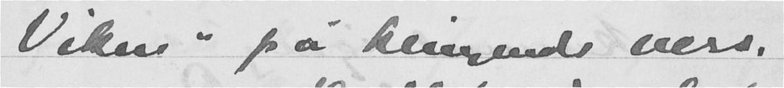Viken" på klingends vers.
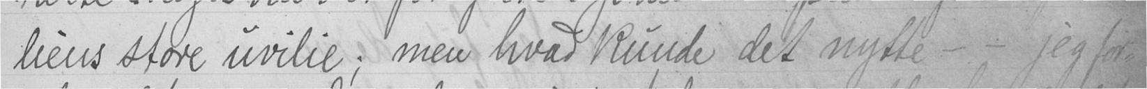liens store uvilie; men hvad kunde det nytte - - jeg for-
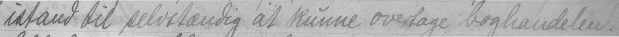istand til selvstændig at kunne overtage boghandelen.
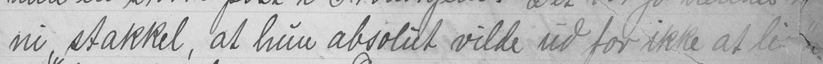ni, stakkel, at hun absolut vilde ud for ikke at li
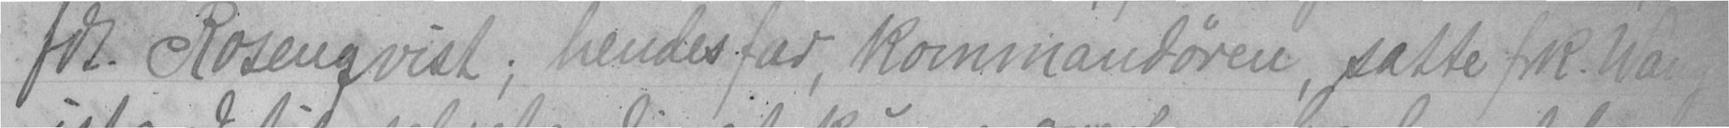fdt. Rosenqvist; hendes far, kommandøren, satte frk. Wang
